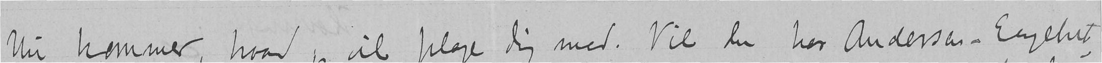Nu kommer, hvad jeg vil plage dig med. Vil du hos Andersen-Engebret-
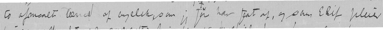to afmaalt lærred af engelck, som jeg før har faat af, og som Eilif pleier
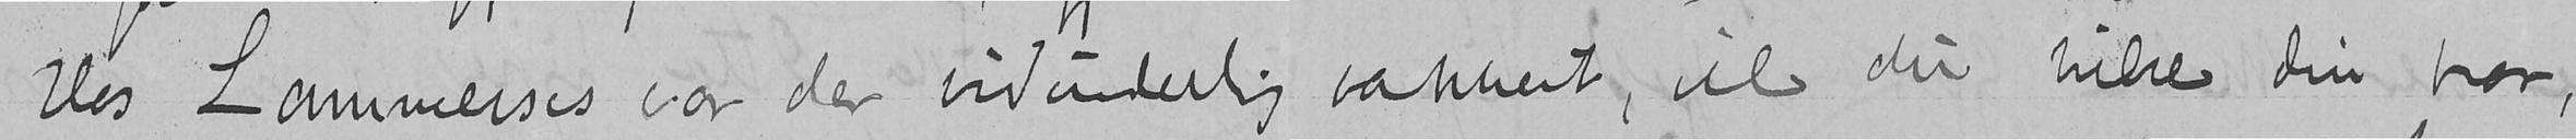Hos Lammerses var der vidunderlig vakkert, vil du hilse din bror,
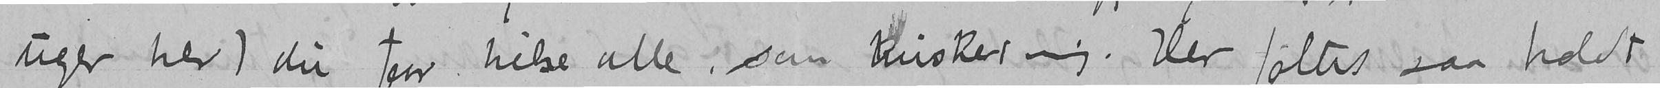uger her) du faar hilse alle, som husker mig. Her føltes saa koldt
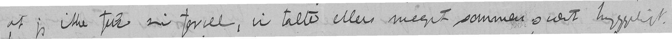at jeg ikke fik si farvel, vi talte ellers meget sammen svært hyggeligt.
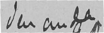den andre
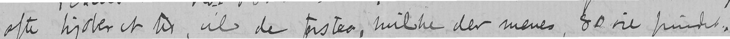ofte kjøber et der, vil de forstaa, hvilke der menes, og vil findes.
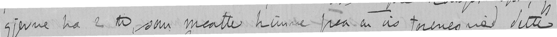gjerne ha 2 til, som maatte kunne paa en vis forenes med dette
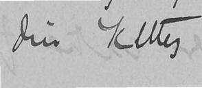din Kitty
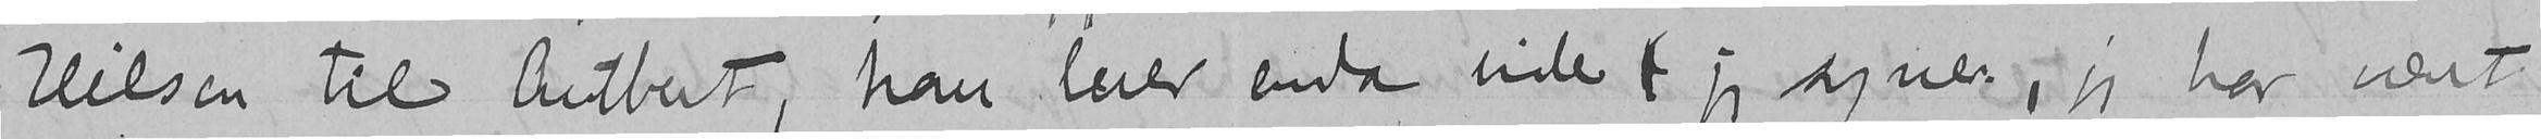Hilsen til Aubert, han lever enda ude (jeg synes, jeg har vært
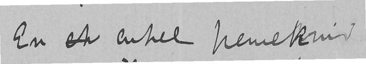Eu ek enkel pennekniv
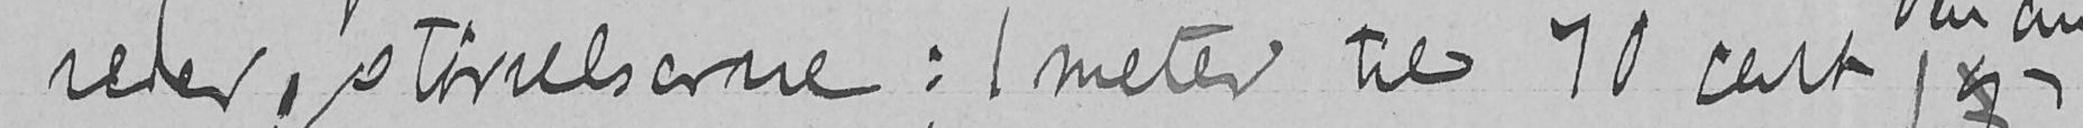reder, størrelserne: 1 meter til 10 cent, og,
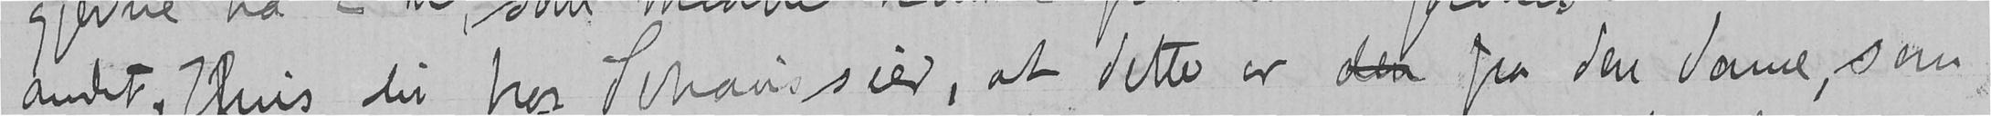andet. Hvis du hos Schau sier, at dette er den fra den dame, som
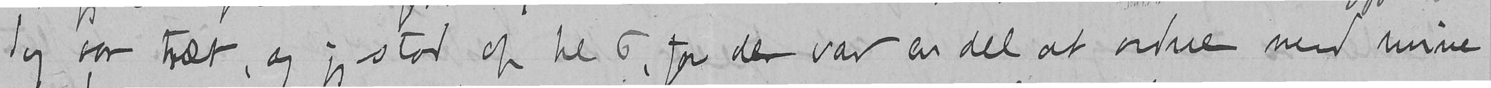Jeg var træt, og jeg stod op kl 6, for der var en del at ordne med mine
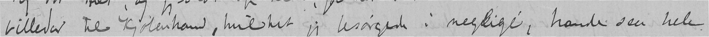billeder til Kjøbenhavn, hvilket jeg besørgede i negligé, havde saa hele
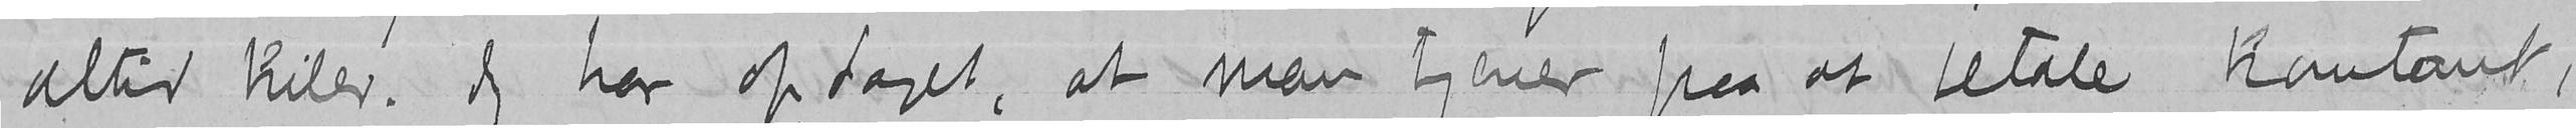altid kiler. Jeg har opdaget, at man tjener paa at betale kontant,
